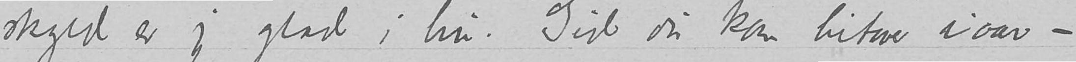skyld er jeg glad i hu. Gid du kom hitover ivaar -
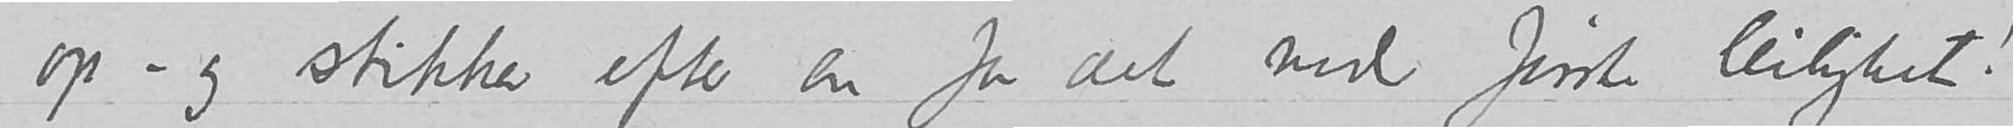op - og stikker efter en for det ved første leilighet!
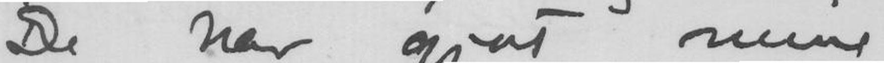De har gjort mine
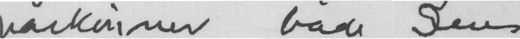påskønner både Deres
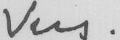Vers.
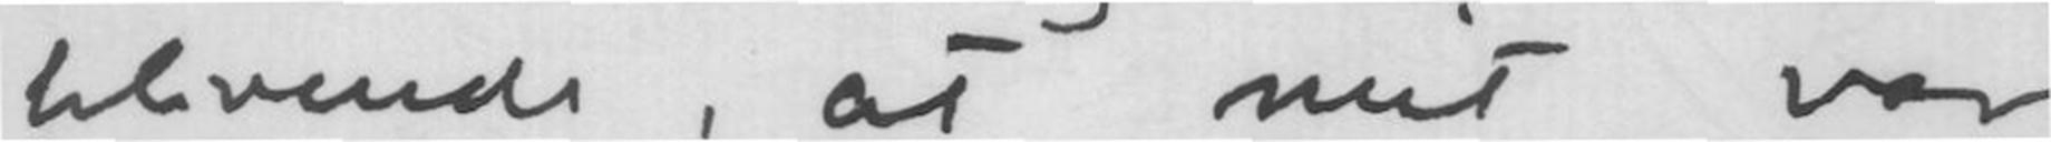blivende, at mit var
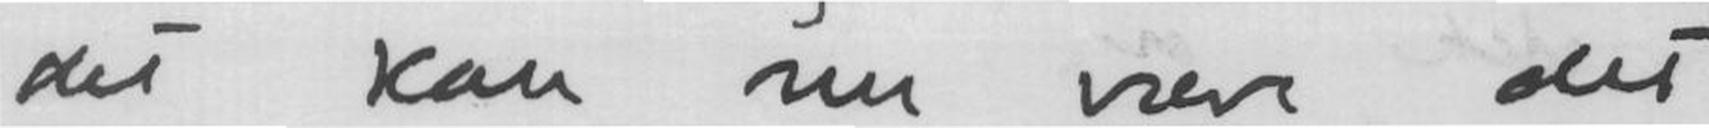det kan nu være det
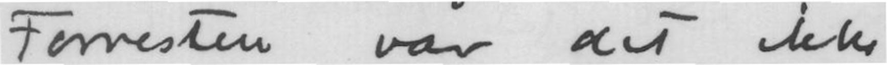Forresten var det ikke
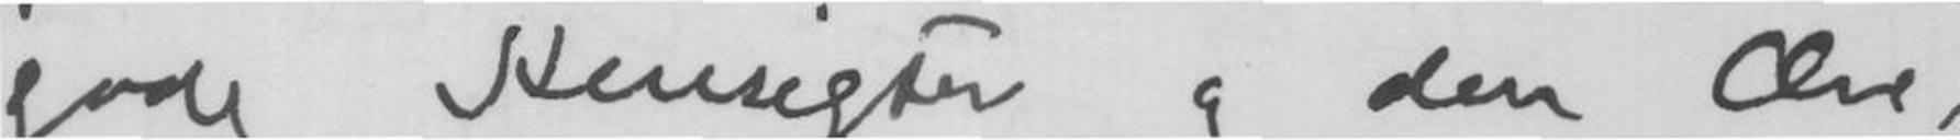gode Hensigter og den Ære,
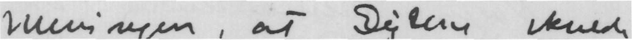Meningen, at Digtene skulde
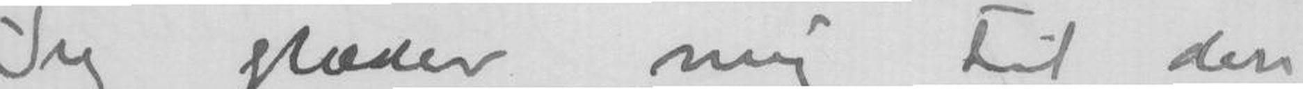Jeg glæder mig til den
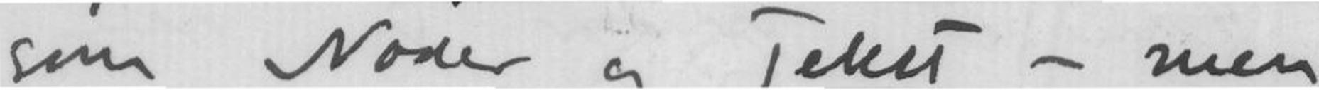som Noder ogf Tekst - men
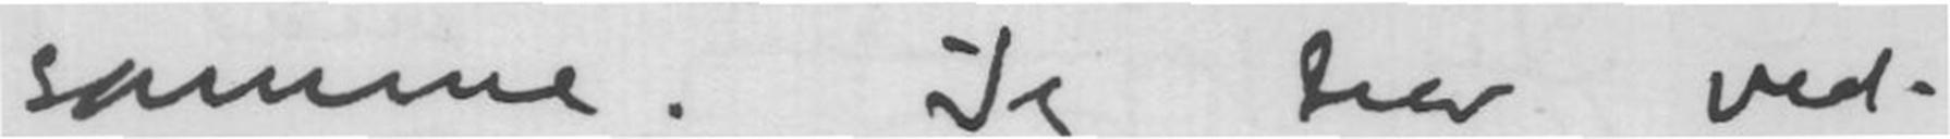samme. Jeg tror ved-
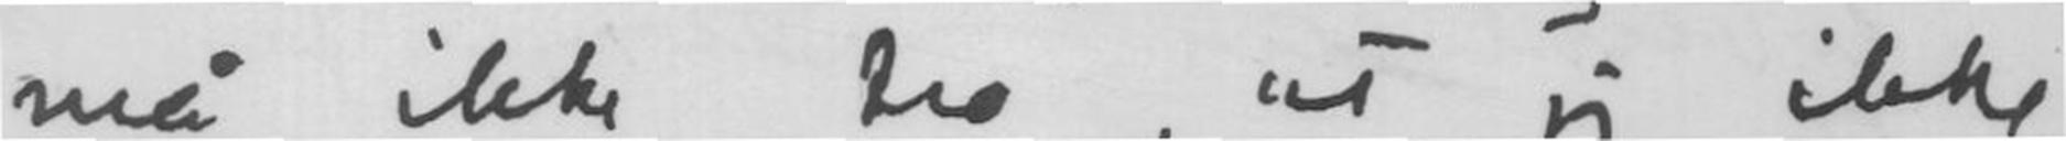må ikke tro, at jeg ikke
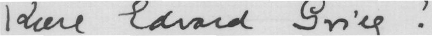Kære Edvard Grieg!
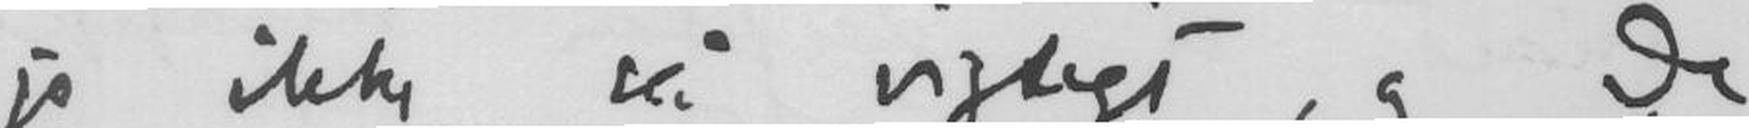jo ikke så vigtigt, og De
